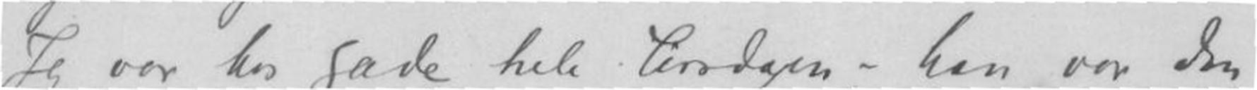Jeg var hos Gade hele tirsdagen, - han var den
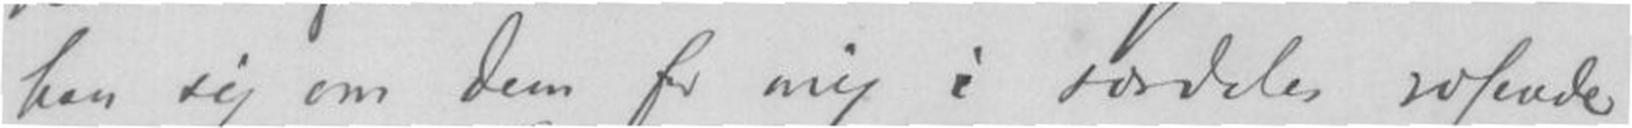han sig om Dem for mig i særdeles rosende
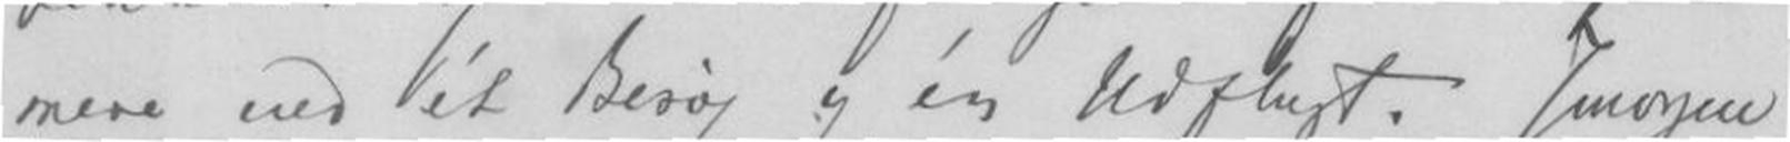mere end et Besøg og en Udflygt. Imorgen
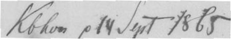Kbhvn p 14 Sept 1885??
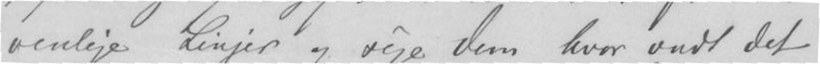venlige Linjer og sige Dem hvor vondt det
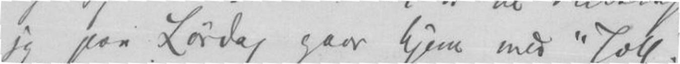jeg paa Lørdag gaar hjem med "Toll".
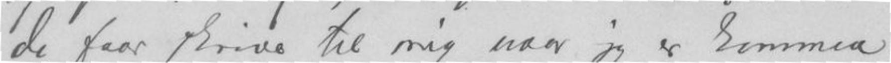De faar skrive til mig naar jeg er kommen
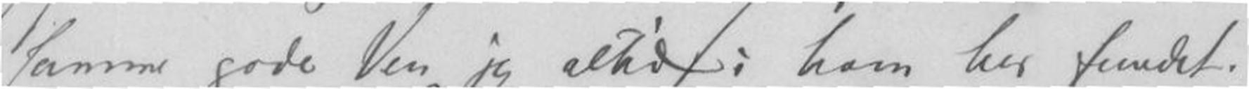samme gode Ven jeg altid i ham har fundet.
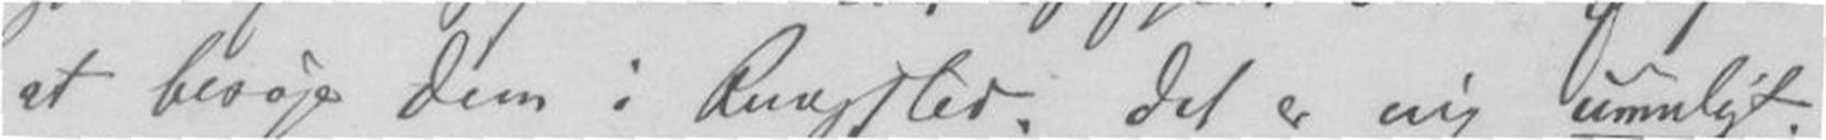at besøge Dem i Rungsted??. Det er mig umuligt.
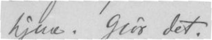hjem. Gjør det.
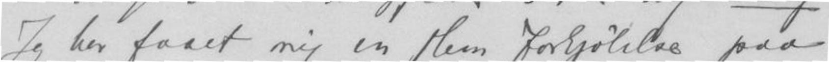Jeg har faaet mig en slem forkjølelse paa
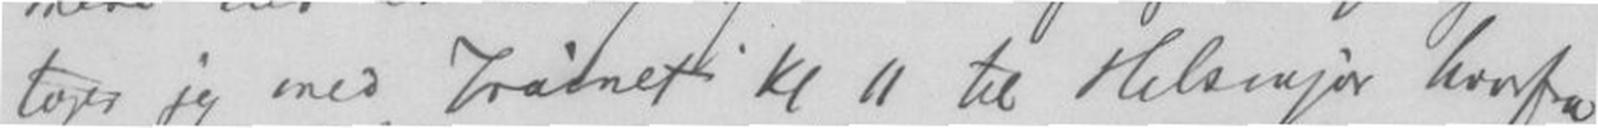tages jeg med Trådnet Kl 11 til Helsingør hvorfra
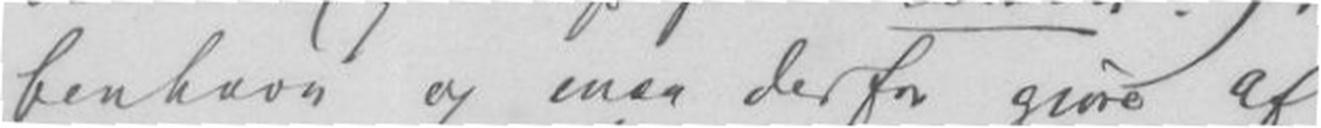benhavn og maa derfor gjøre af
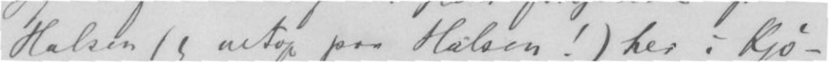Halsen (og netop paa Halsen !) her i Kjø-
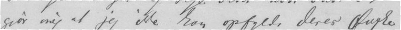gjør mig at jeg ikke kan opfylde Deres Ønske
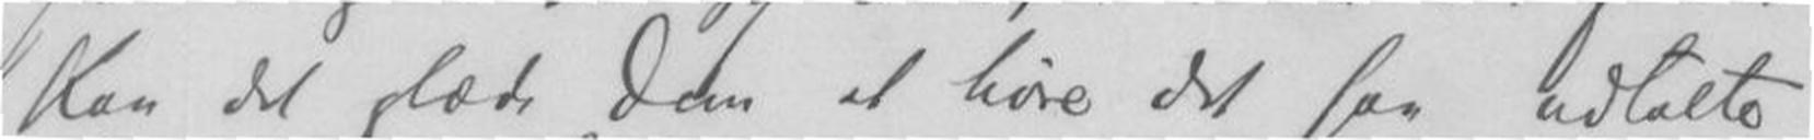Kan det glæde Dem at høre det saa udtalte
